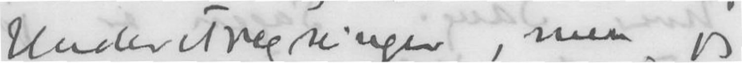Understregninger, men jeg
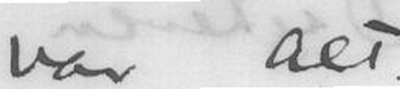var Alt.
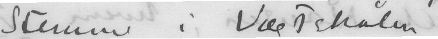Stemmer i Vægtskålen.
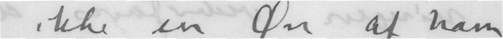jo ikke en Øre af ham
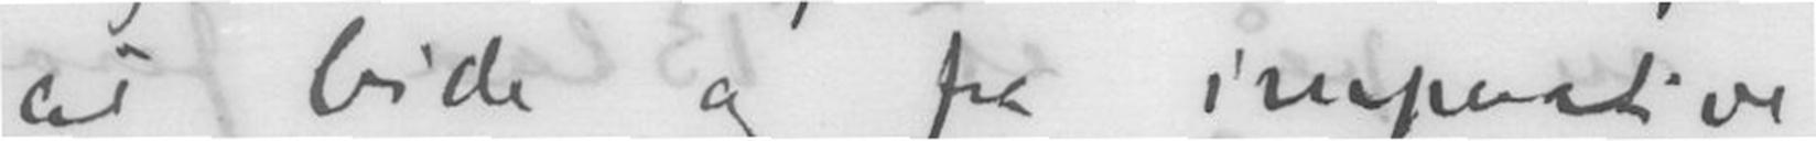at bide og fra imperative
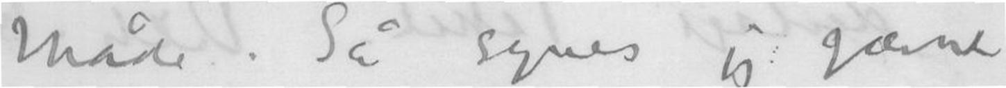Måde. Så synes jeg gærne
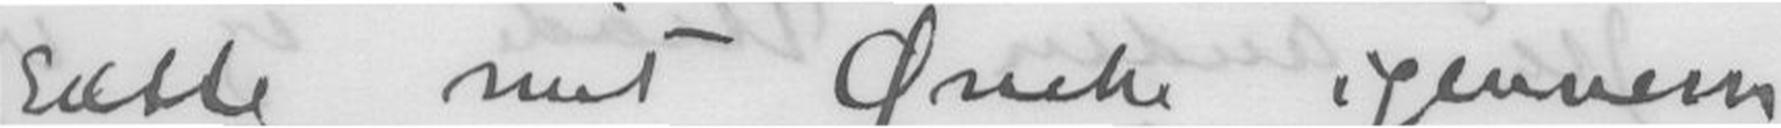sætte mit Ønske igennem
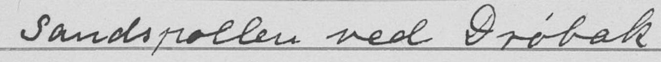Sandspollen ved Drøbak
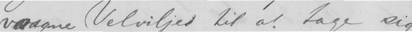vaagne Velvilje til at tage sig
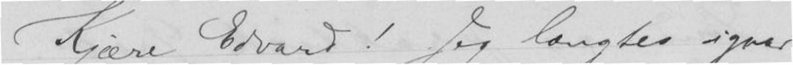Kjære Edvard! Jeg længtes igaar
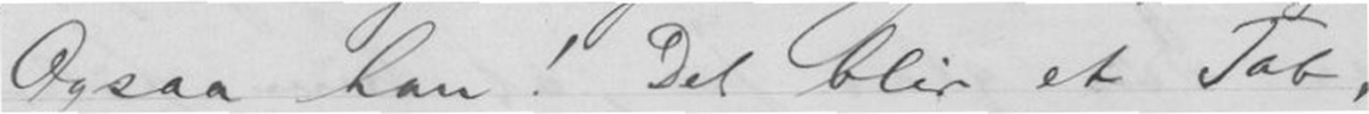Ogsaa han! Det blir et Tab,
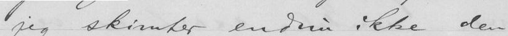jeg skimter endnu ikke den
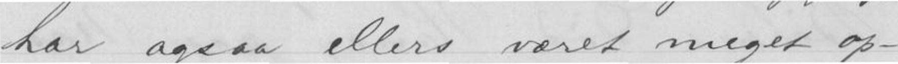har ogsaa ellers været meget op-
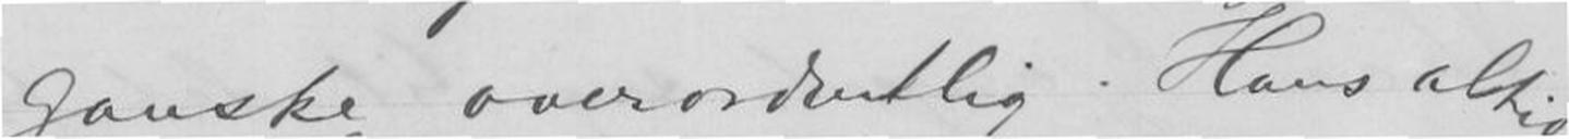ganske overordentlig. Hans altid
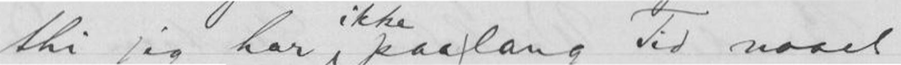thi jeg har paa lang Tid naaet
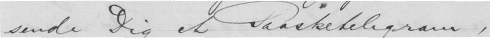sende Dig et Paasketelegram,
